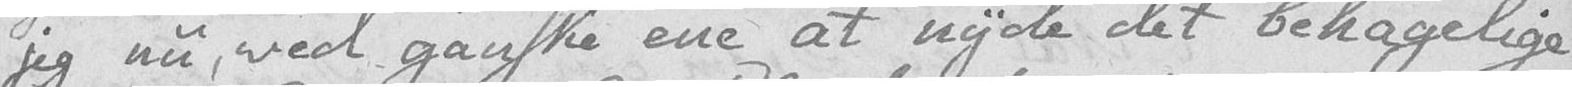jeg nu, ved ganske ene at nyde det behagelige
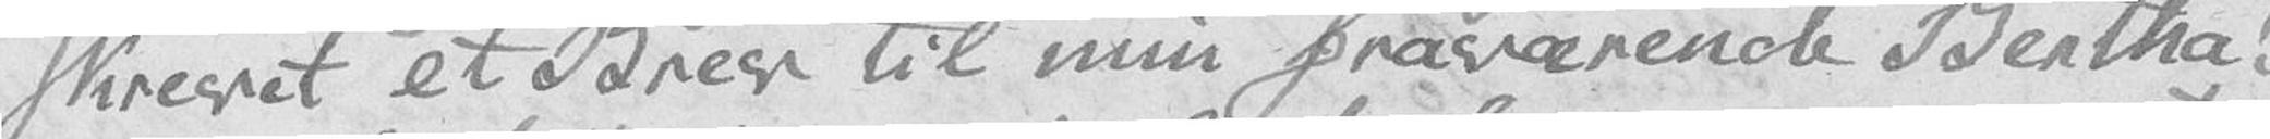skrevet et Brev til min fraværende Bertha!
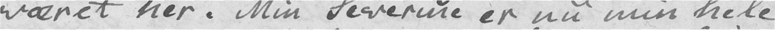været her. Min Severine er nu min hele
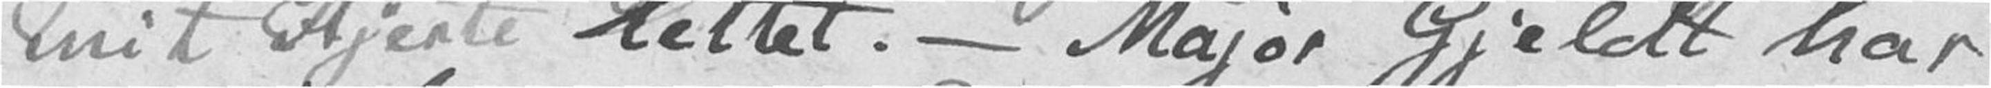mit Hjerte lettet. – Major Gjeldt har
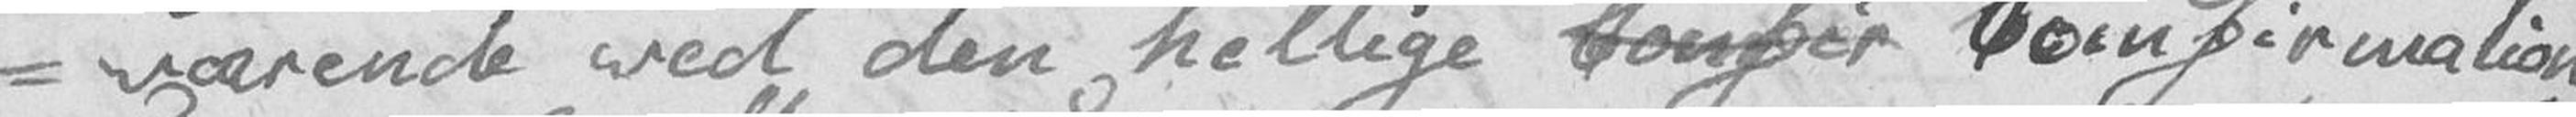-værende ved den hellige Comfir Comfirmation
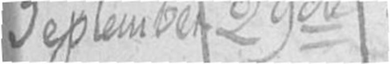September 29de
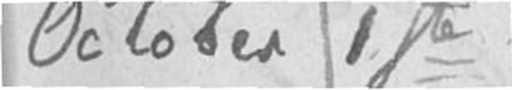October 1ste
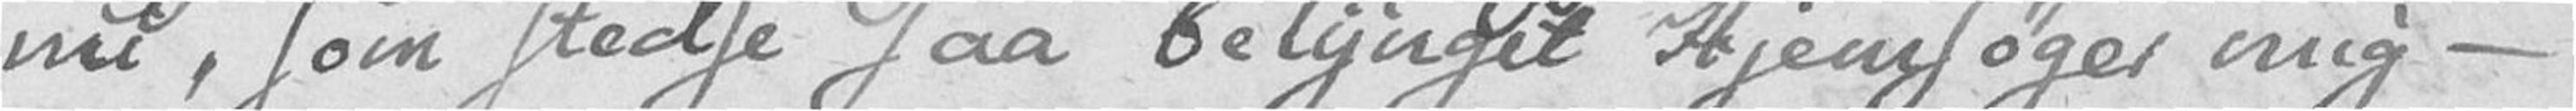nu, som stedse saa betynget Hjemsøger mig –
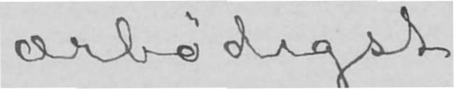ærbødigst
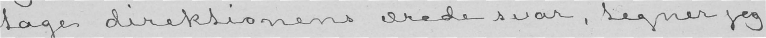tage direktionens ærede svar, tegner jeg
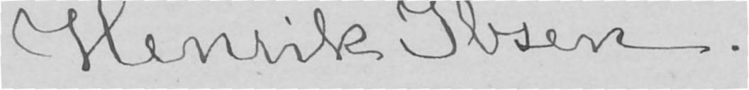Henrik Ibsen.
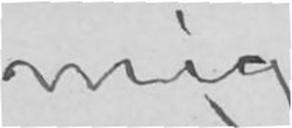mig
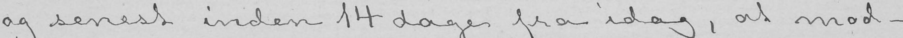og senest inden 14 dage fra idag, at mod-
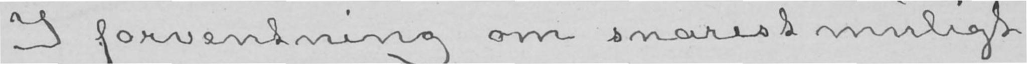I forventning om snarest muligt,
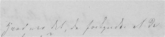Hvad var det, de forkyndte at de
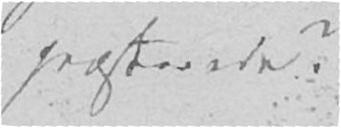præsterede?
